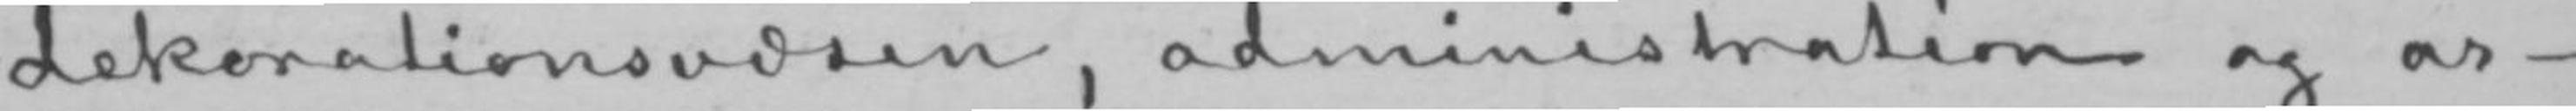dekorationsvæsen, administration og ar-
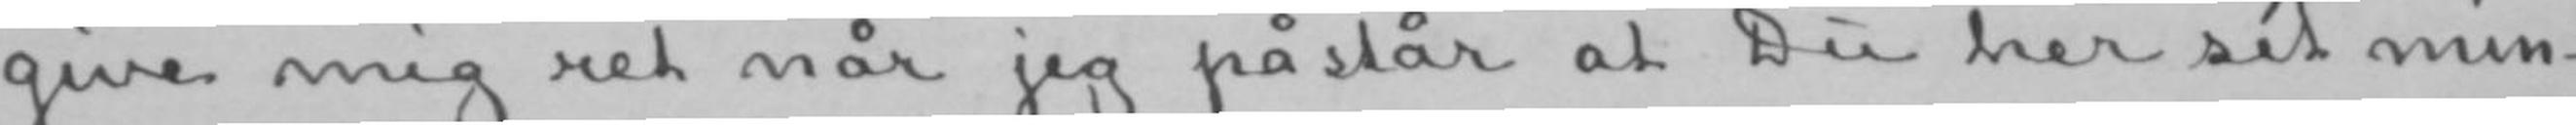give mig ret når jeg påstår at Du har sét min-
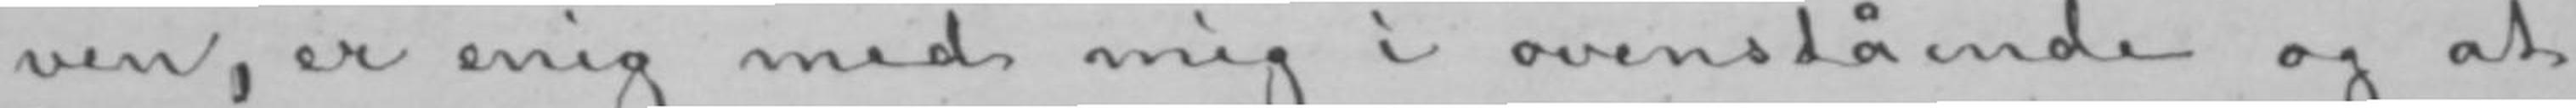ven, er enig med mig i ovenstående og at
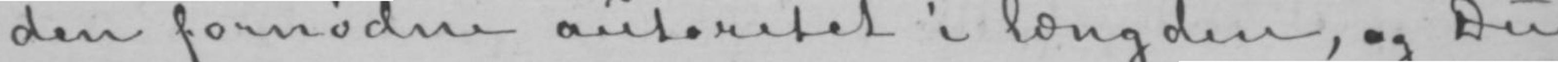den fornødne autoritet i længden, og Du
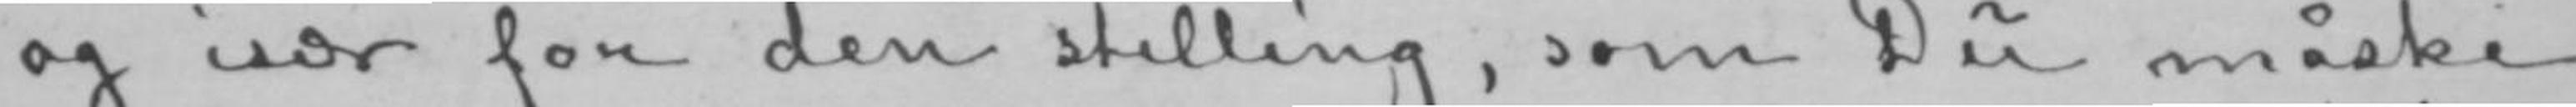og især for den stilling, som Du måske
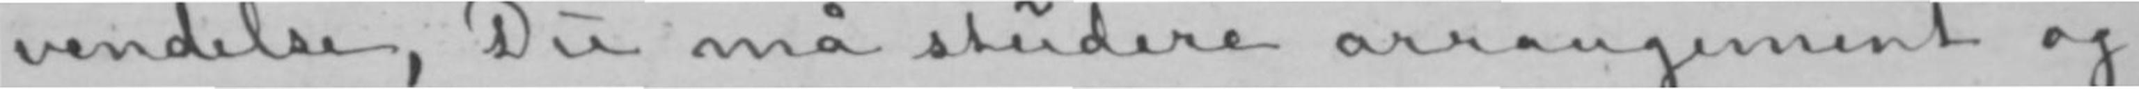vendelse, Du må studere arrangement og
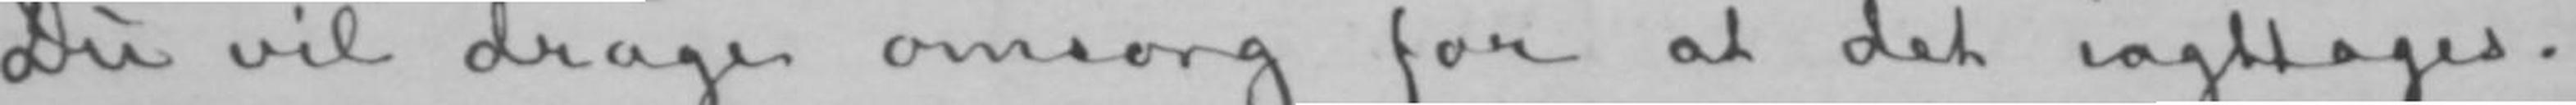Du vil drage omsorg for at det iagttages.-
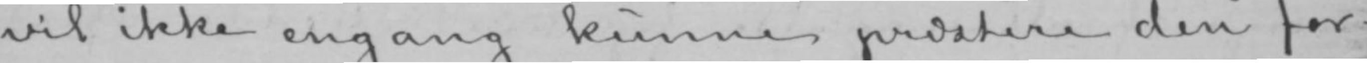vil ikke engang kunne præstere den for-
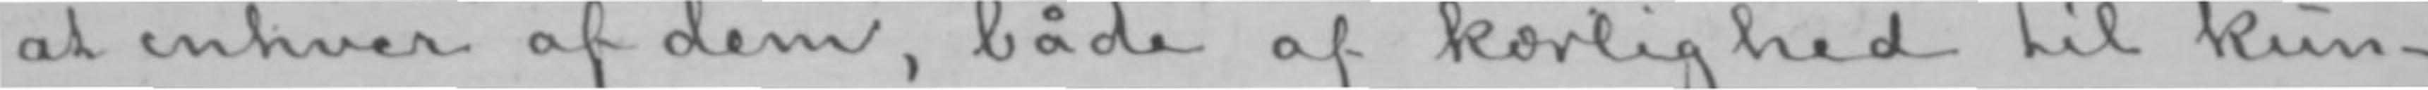at enhver af dem, både af kærlighed til kun-
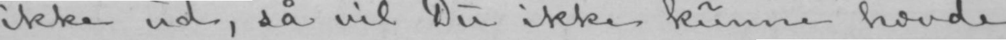ikke ud, så vil Du ikke kunne hævde
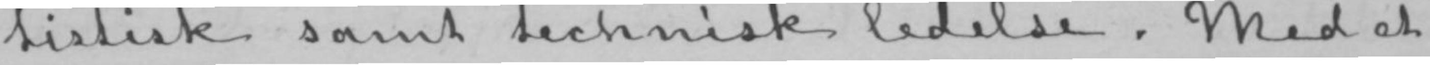tistisk samt technisk ledelse. Med et
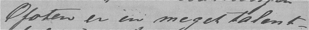Ofoten er en meget talent-
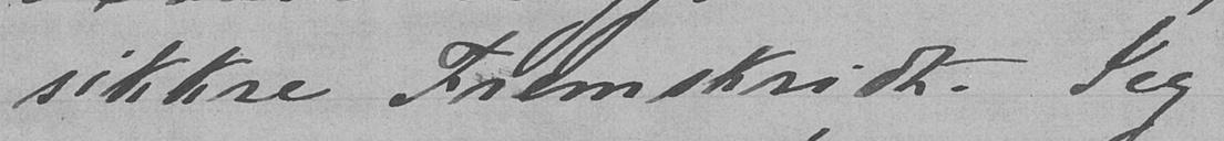sikkre Fremskridt. Jeg
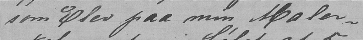som Elev paa min Maler-
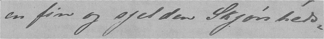en fin og sjelden Skjønheds-
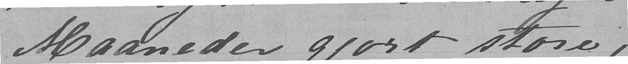Maaneder gjort store,
